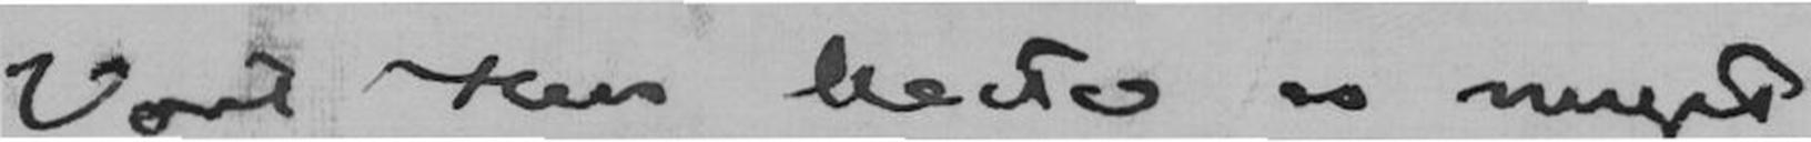Vort Hus koster os meget
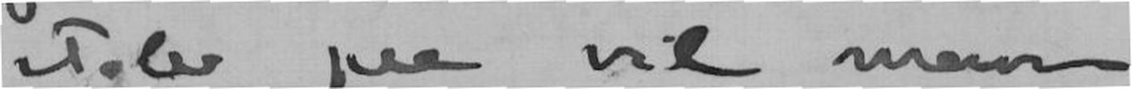stoler paa vil man
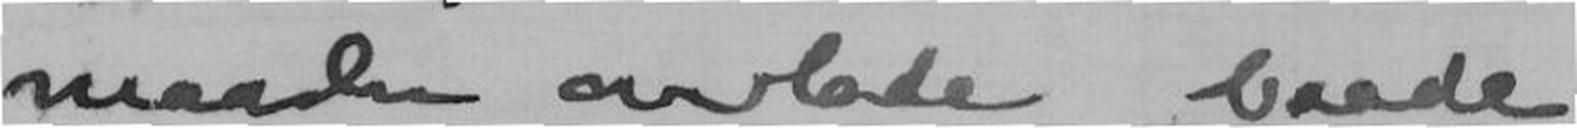maaske avlade baade
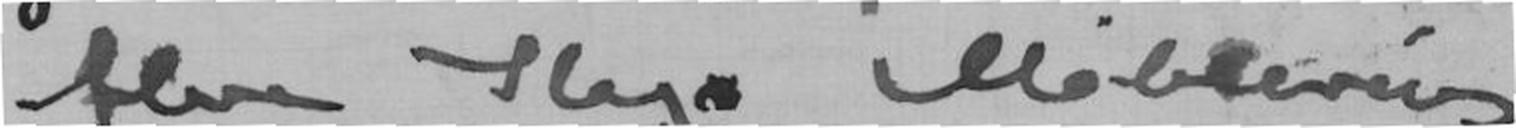flere slags Møblering
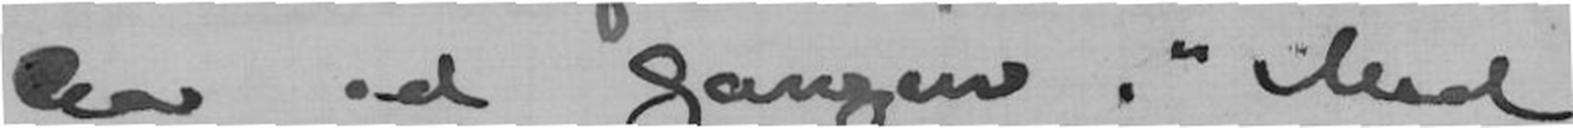Aar ad Gangen. "Med
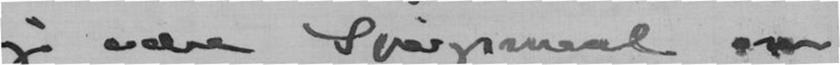jo være Spørgsmaal om
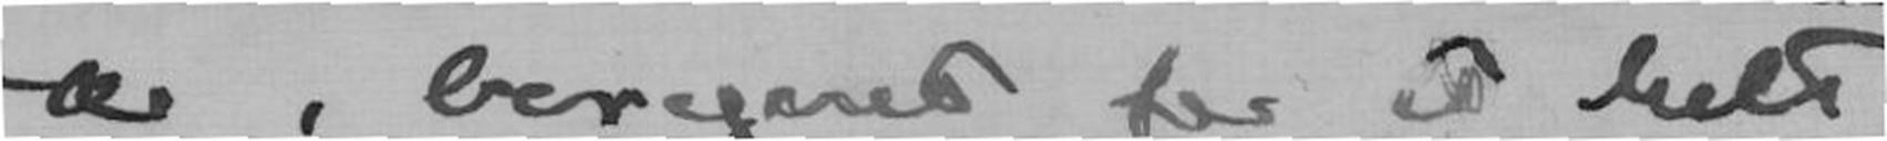Kr, beregnet for et helt
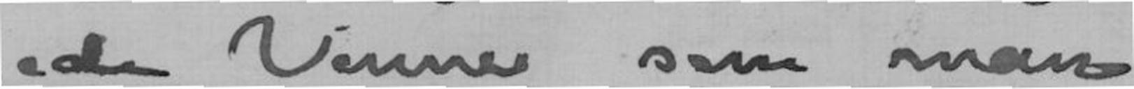gode Venner som man
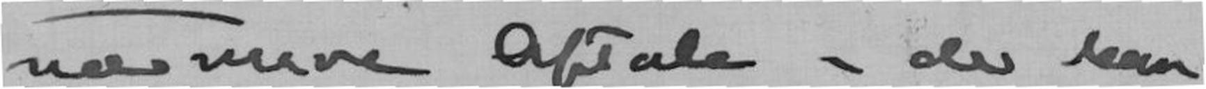nærmere Aftale - der kan
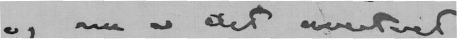og nu er det averteret
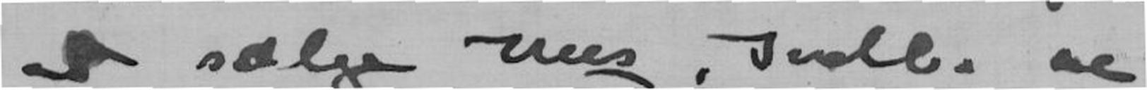at sælge Hus. Indbo etc.
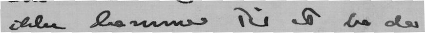ikke Sommer Til at bo der
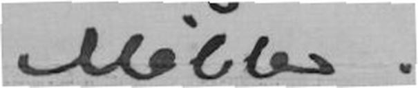Møbler.
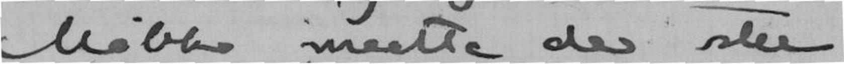Møbler maatte der ske
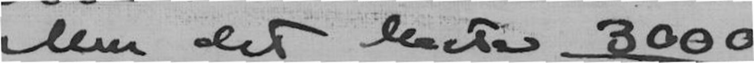Men det koster 3000
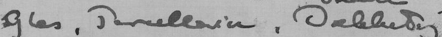Glas, Porcellain, Dækketøj
